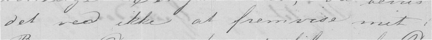det ved ikke at fremvise mit
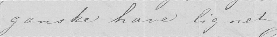ganske have lignet
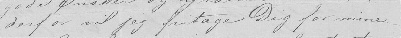derfor vil jeg fritage Dig for mine. -
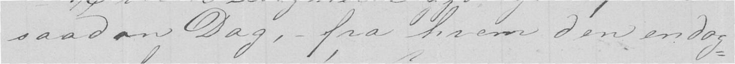saadan Dag, - fra hvem den endog-
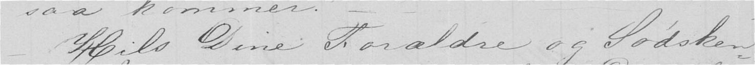- Hils Dine Forældre og Sødsken-
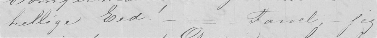hellige Eed! - - - Farvel, - jeg
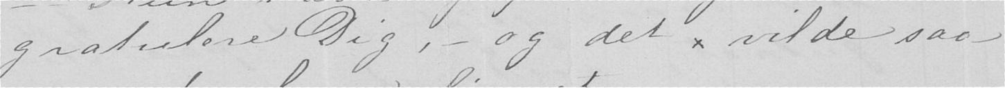gratulere Dig, - og det, vilde saa
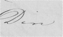Din
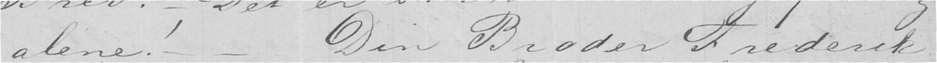alene! - - Din Broder Frederik
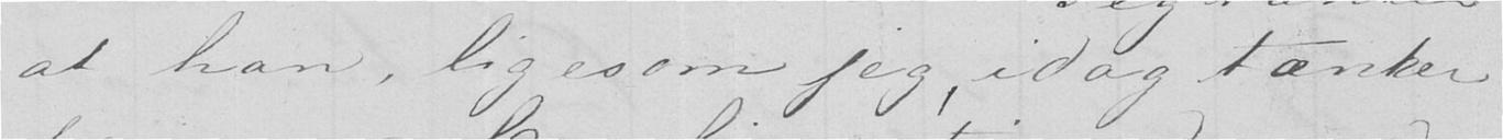at han, ligesom jeg, idag tænker
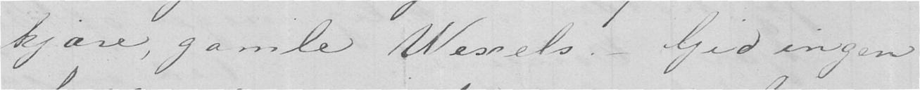kjære, gamle Wexels. - Gid ingen
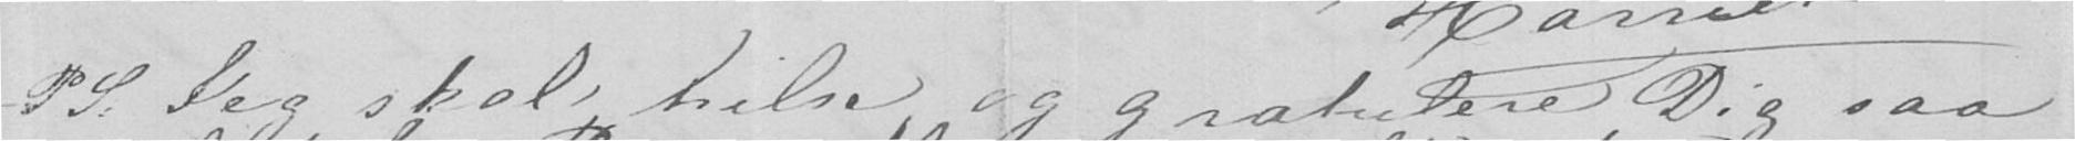PS. Jeg skal hilse og gratulere Dig saa
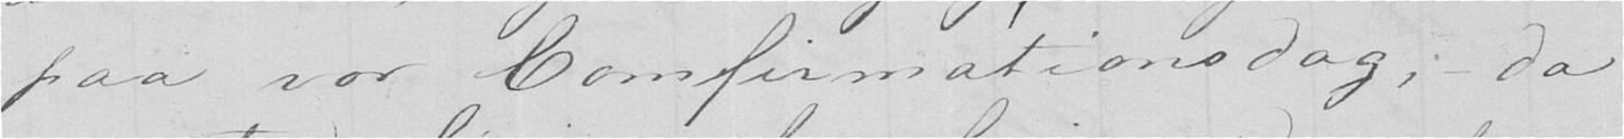paa vor Comfirmationsdag, - da
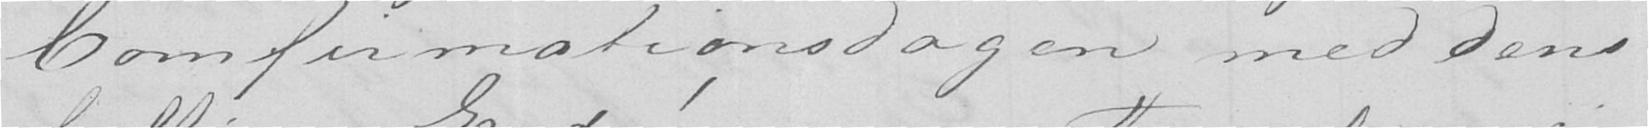Comfirmationsdagen med dens
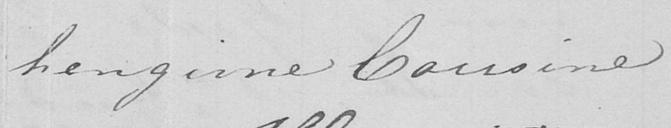hengivne Cousine
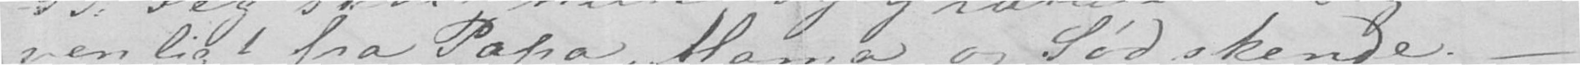venligt fra Papa, Mama og Sødskende. -
